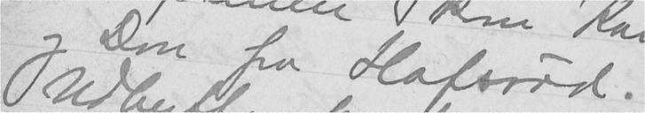og Don fra Hafsrød.
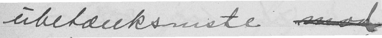ubetænksomste mod
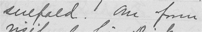snefald. Om form.
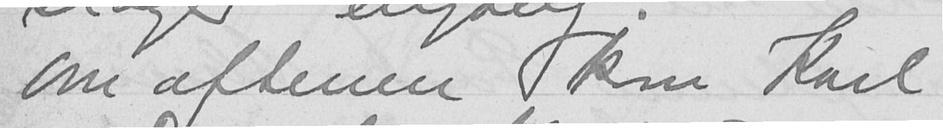Om aftenen kom Karl
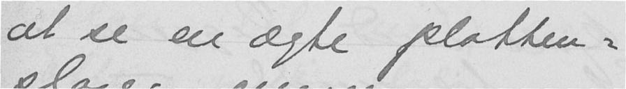at se en ægte platten-
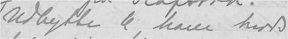Udbytte 6 harer trods
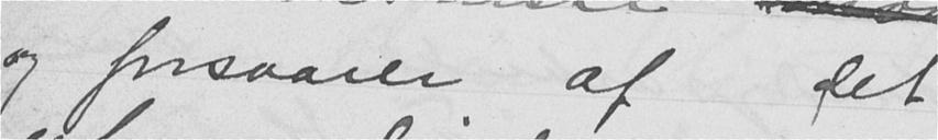og forsvarer af det
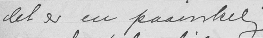det er en paavirkelig
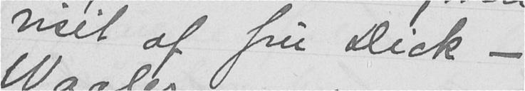visit af fru Dick-
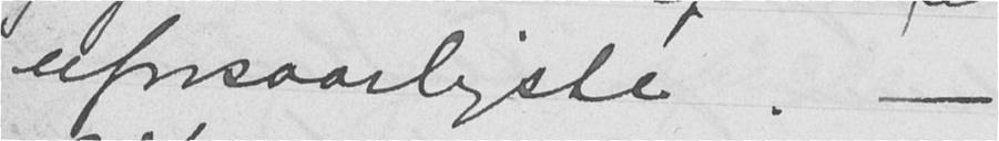uforsvarligste. -
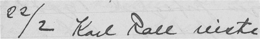22/2 Karl Roll reiste
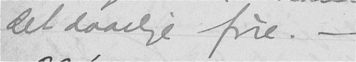det daarlige føre. -
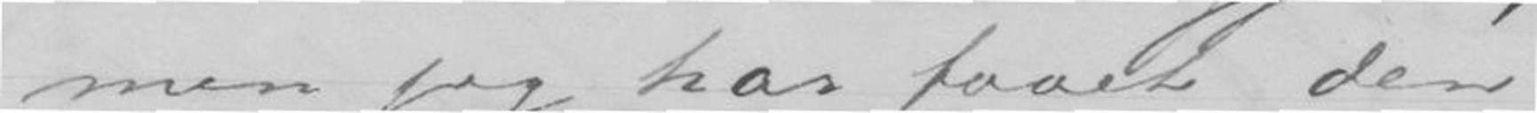men jeg har faaet den
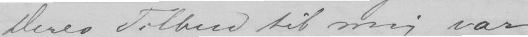Deres Tilbud til mig var
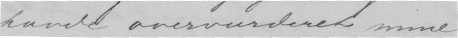havde overvurderet mine
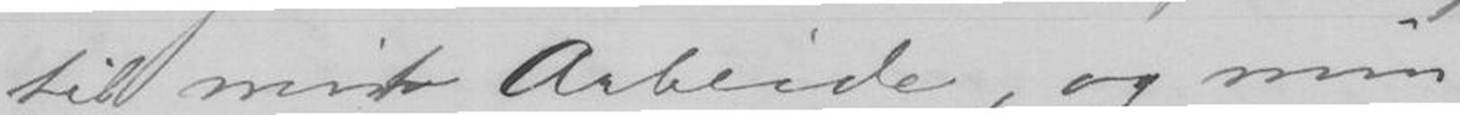til mit Arbeide, og min
